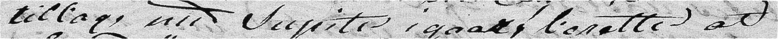tilbage med Jupiter igaar, beretter at
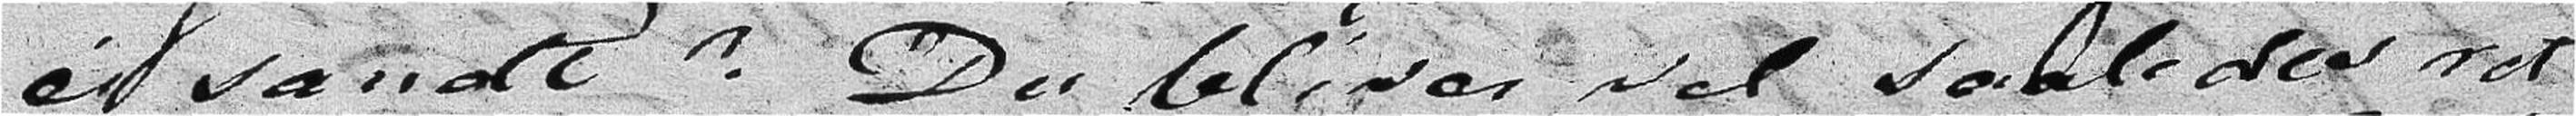ei sandt? Du bliver vel saaledes ret
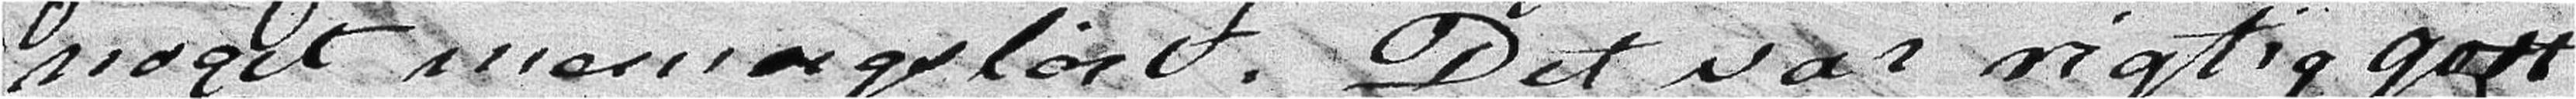noget meningsløst. Det var rigtig godt
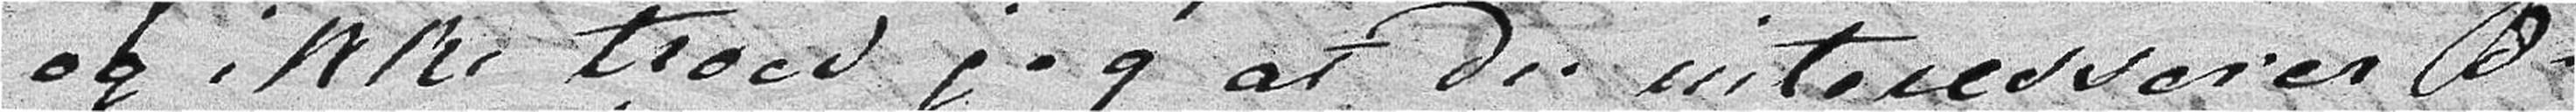og ikke troer jeg at Du interesserer Dig
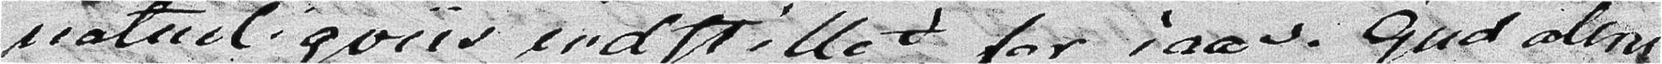naturligviis indstillet for iaar. Gud alene
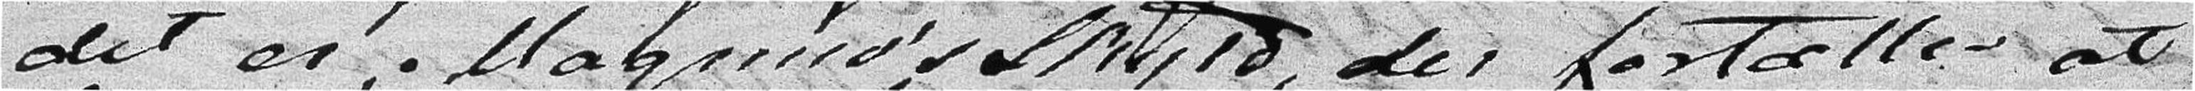der er, Magnus Skyld, der fortæller at
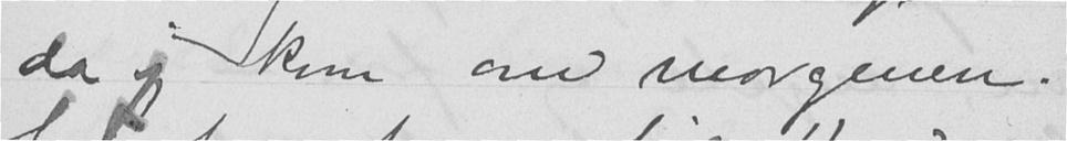da jeg kom om morgenen.
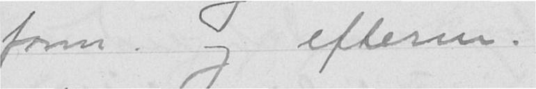form. og efterm.
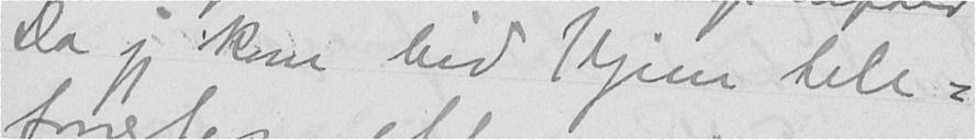Da jeg kom hid hjem tele-
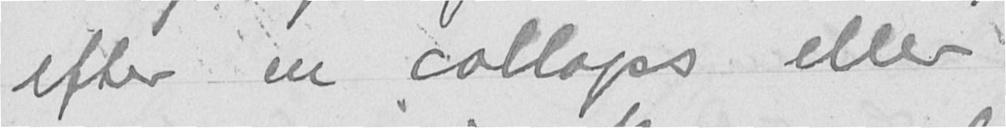efter en collaps eller
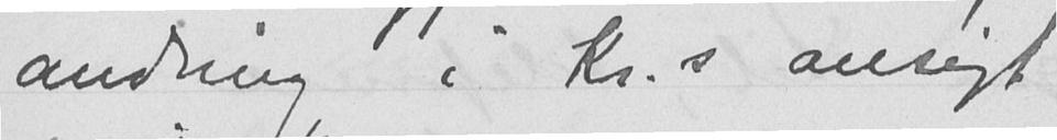andring i Kr.s ansigt
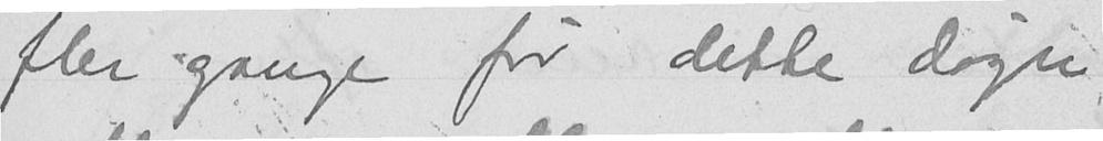fler gange før dette døgn
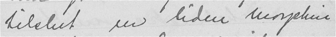tilslut en liden morphin
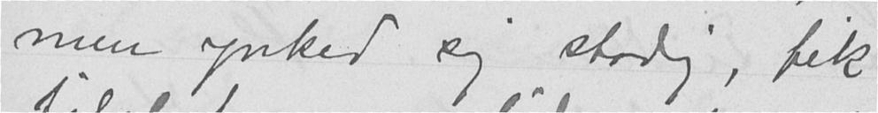men ynked sig stadig, fik
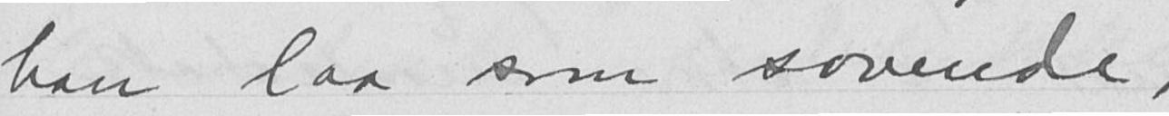han laa som sovende,
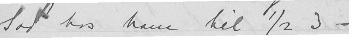Sad hos ham til ½ 3 -
